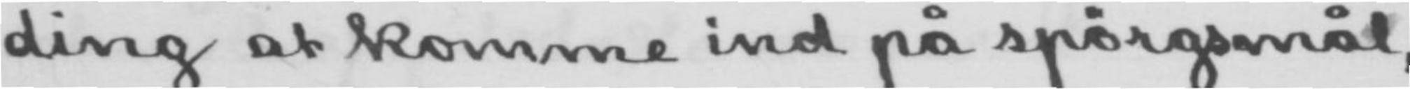ding at komme ind på spørgsmål,
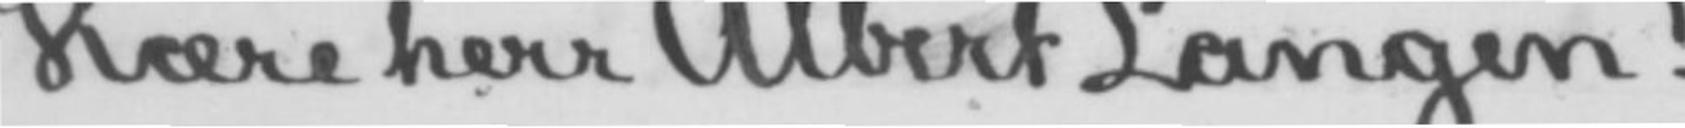Kære herr Albert Langen!
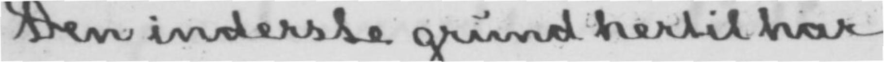Den inderste grund hertil har
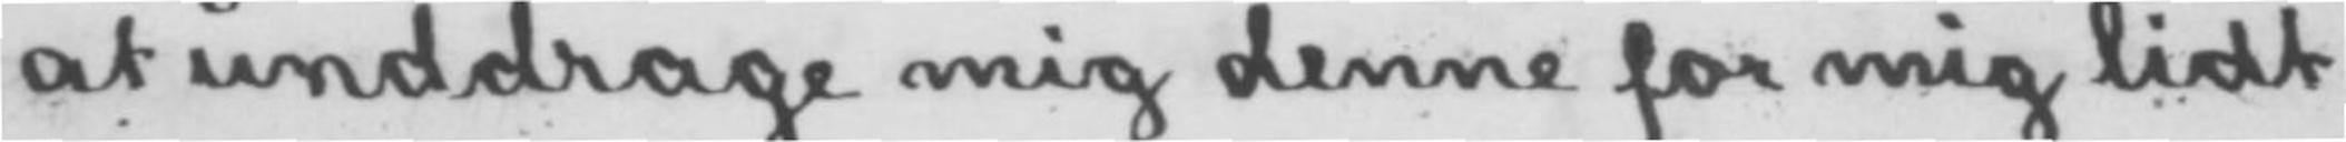at unddrage mig denne for mig lidt
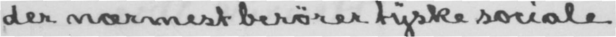der nærmest berører tyske sociale
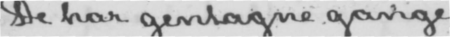De har gentagne gange
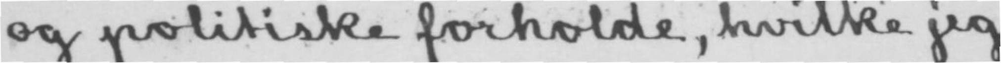og politiske forholde, hvilke jeg
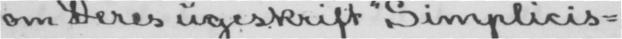om Deres ugeskrift «Simplicis-
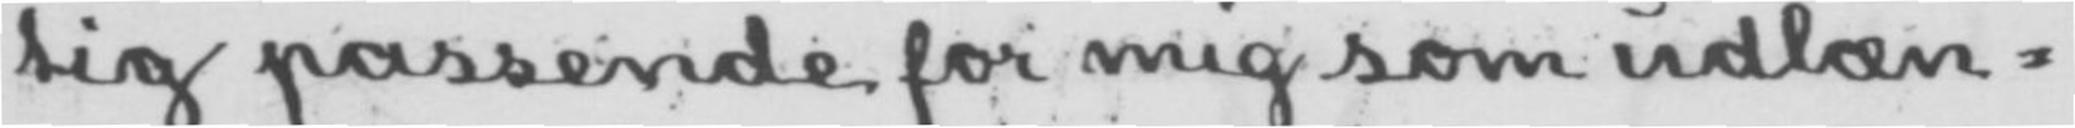tig passende for mig som udlæn-
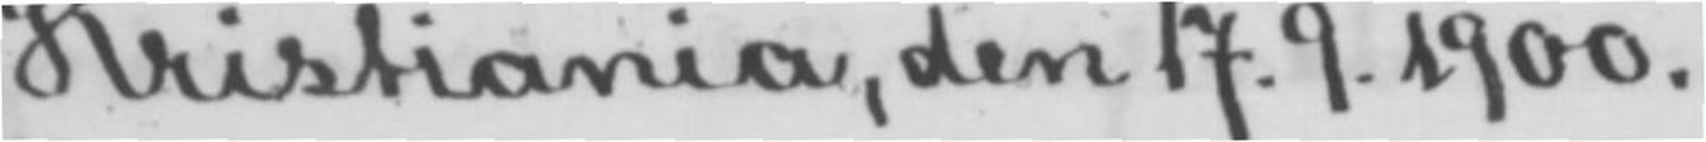Kristiania, den 17.9.1900.
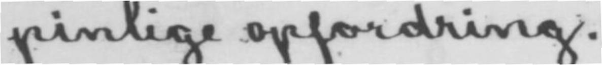pinlige opfordring.
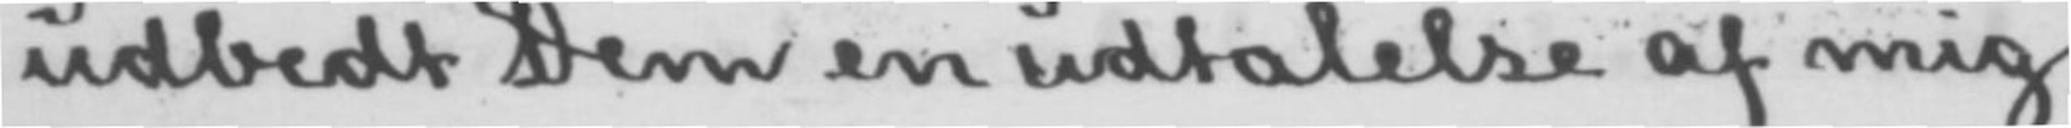udbedt Dem en udtalelse af mig
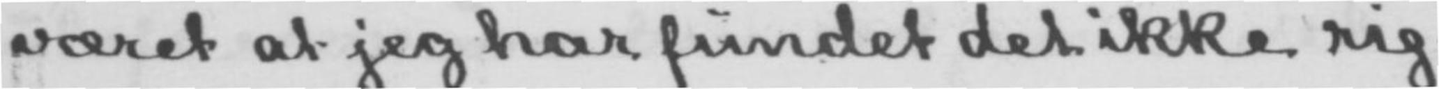været at jeg har fundet det ikke rig-
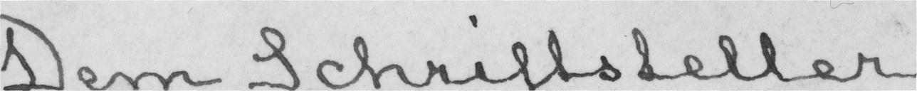Dem Schriftsteller
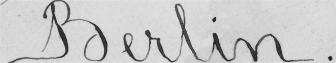Berlin.
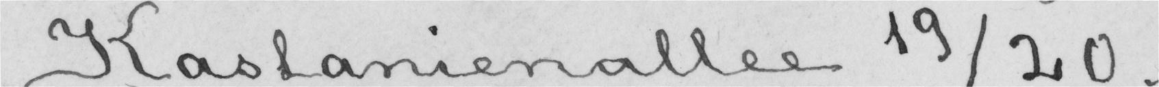Kastanienallee 19&sol;20.
